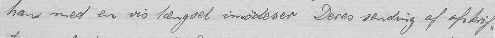han med en vis længsel imødeser Deres sending af afskrif-
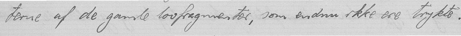terne af de gamle lovfragmente, som endnu ikke ere trykte.
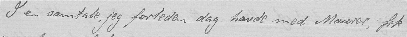I en samtale, jeg forleden dag havde med Maurer, fik
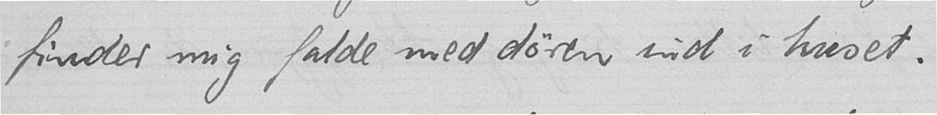finder mig falde med døren ind i huset.
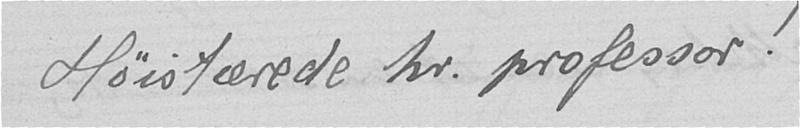Høistærede hr. professor!
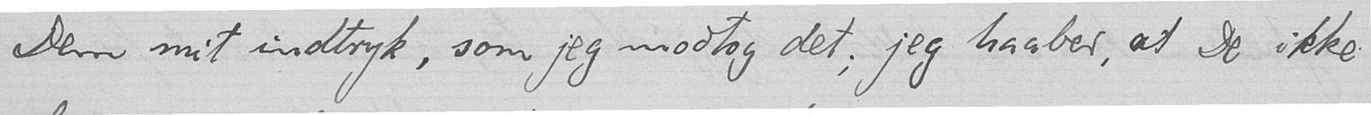Dem mit indtryk, som jeg modtog det; jeg haaber, at De ikke
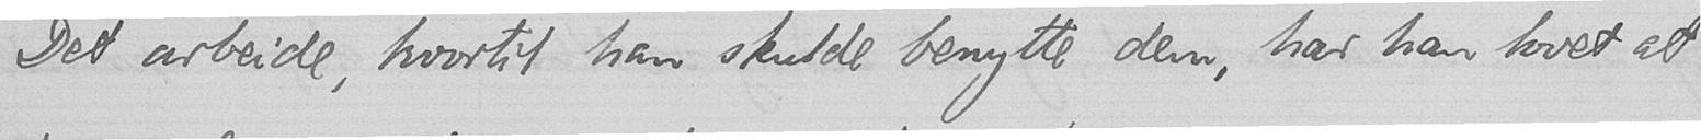Det arbeide, hvortil han skulde benytte dem, har han lovet at
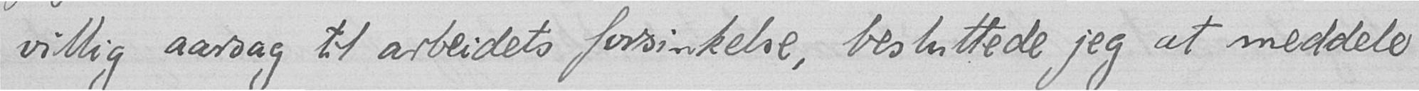villig aarsag til arbeidets forsinkelse, besluttede jeg at meddele
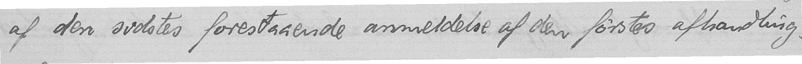af den sidstes forestaaende anmeldelse af den førstes afhandling.
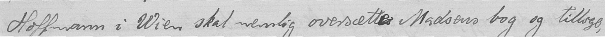Hoffmann i Wien skal nemlig oversætte Madsens bog og tillige,
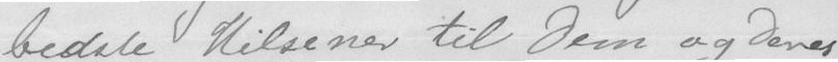bedste Hilsener til Dem og Deres
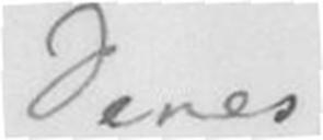Deres
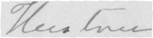Hustru.
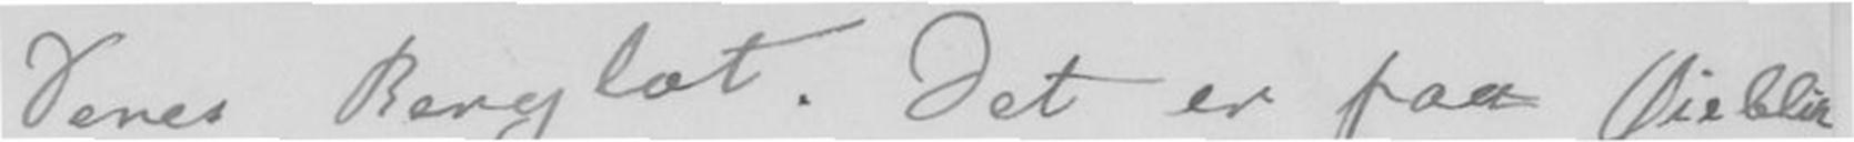Deres Bergliot. Det er faa Øieblik-
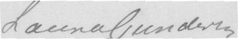Laura Gundersen
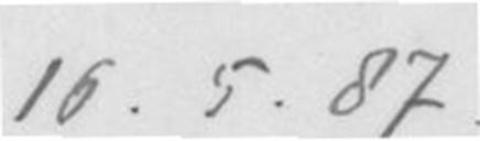16.5.87
